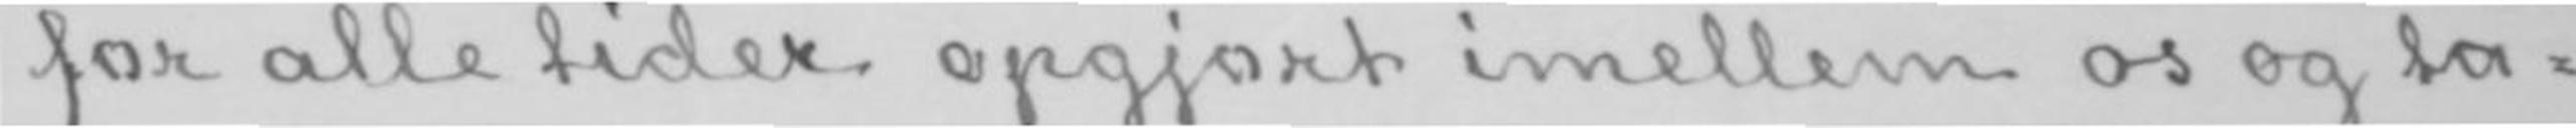for alle tider opgjort imellem os og ta-
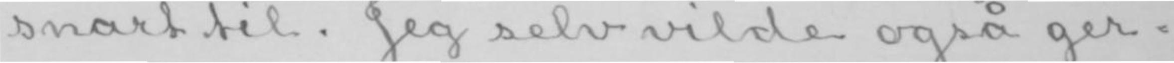snart til. Jeg selv vilde også ger-
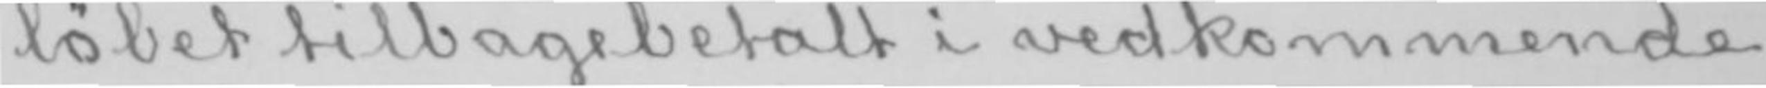løbet tilbagebetalt i vedkommende
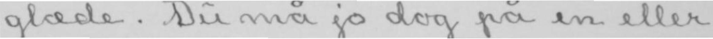glæde. Du må jo dog på en eller
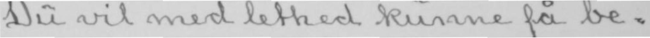Du vil med lethed kunne få be-
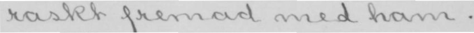raskt fremad med ham.
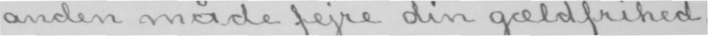anden måde fejre din gældfrihed,
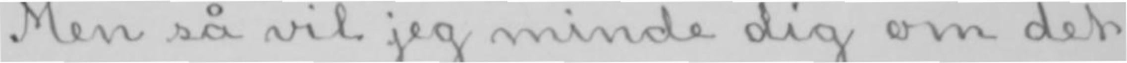Men så vil jeg minde dig om det
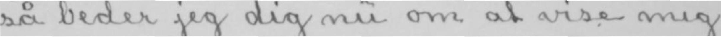så beder jeg dig nu om at vise mig
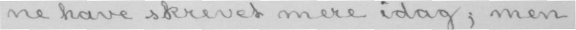ne have skrevet mere idag; men
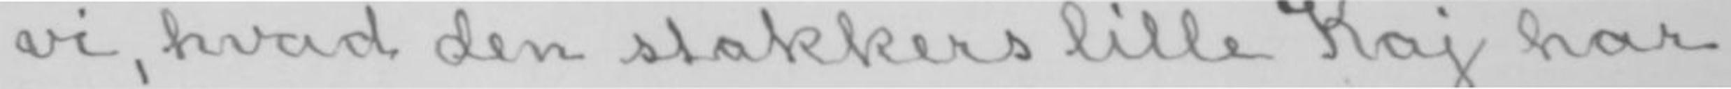vi, hvad den stakkers lille Kaj har
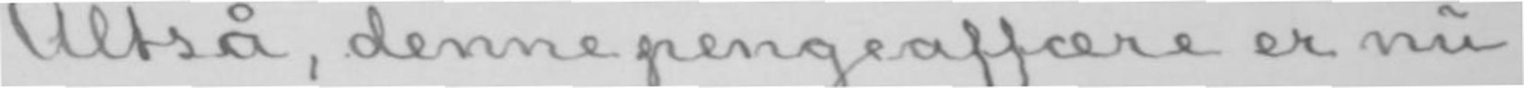Altså, denne pengeaffære er nu
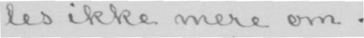les ikke mere om.-
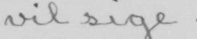vil sige.
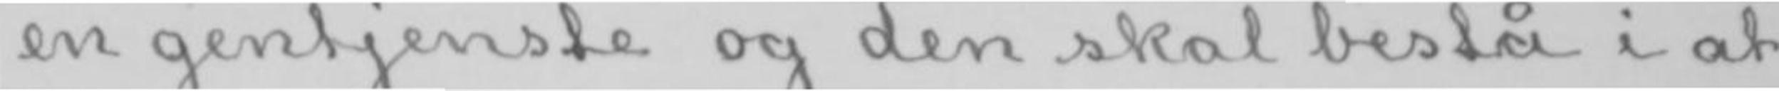en gentjenste og den skal bestå i at
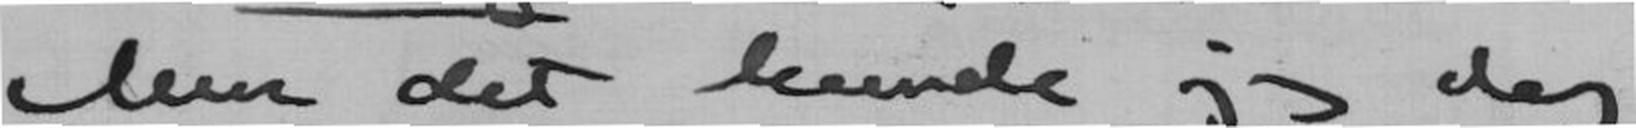Men det kunde jeg dog
